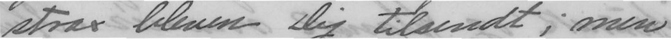strax bleven Dig tilsendt; men
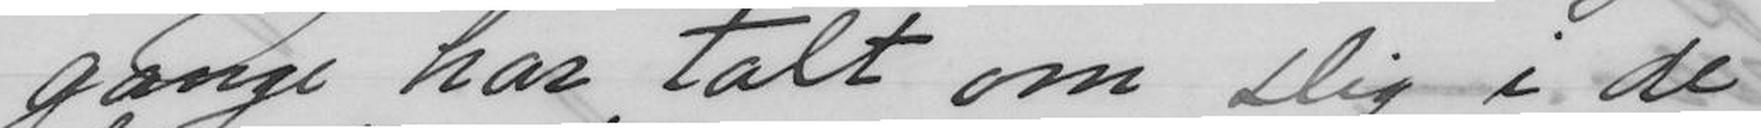gange har talt om Dig i de
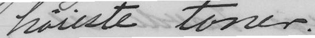høieste toner.
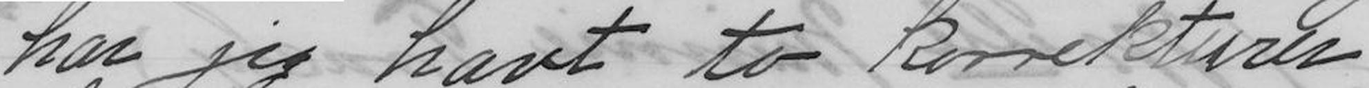har jeg havt to korrekturer
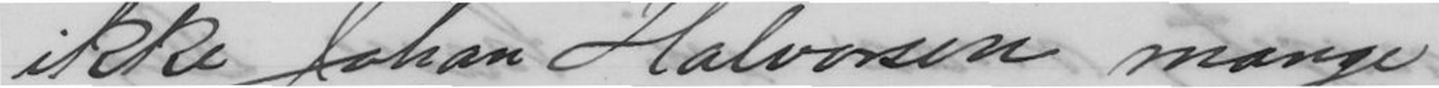ikke Johan Halvorsen mange
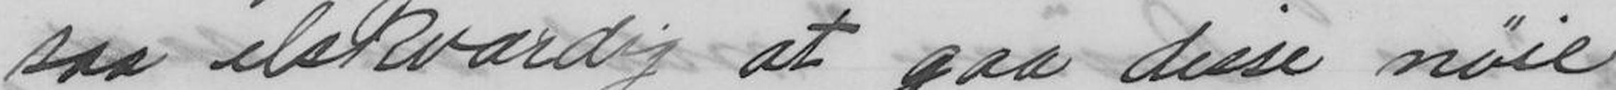saa elskværdig at gaa disse nøie
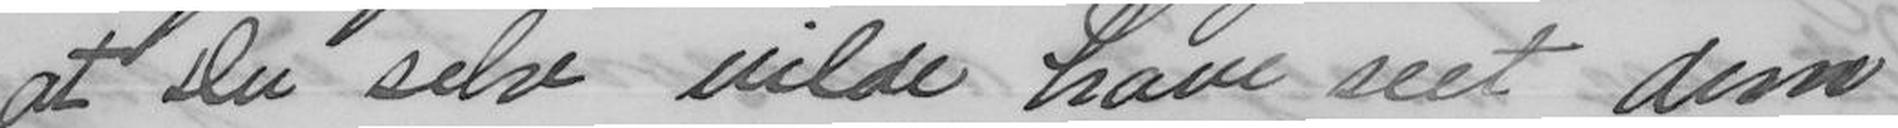at Du selv vilde have seet dem
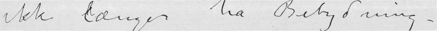ikke længer ha Betydning –
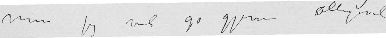men jeg vil jo gjerne allikevel
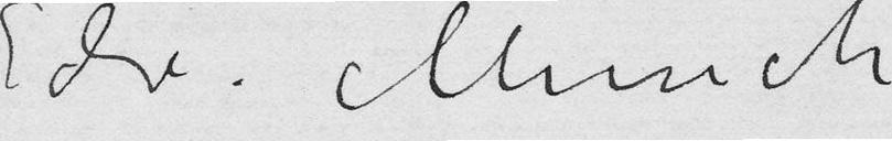Edv. Munch
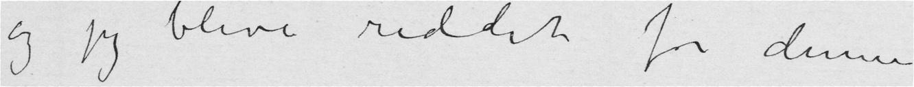og jeg bliver reddet fra denne
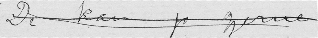De kan jo gjerne
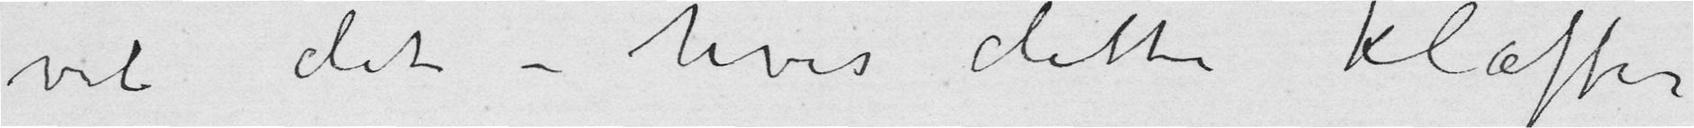vil det – hvis dette klaffer
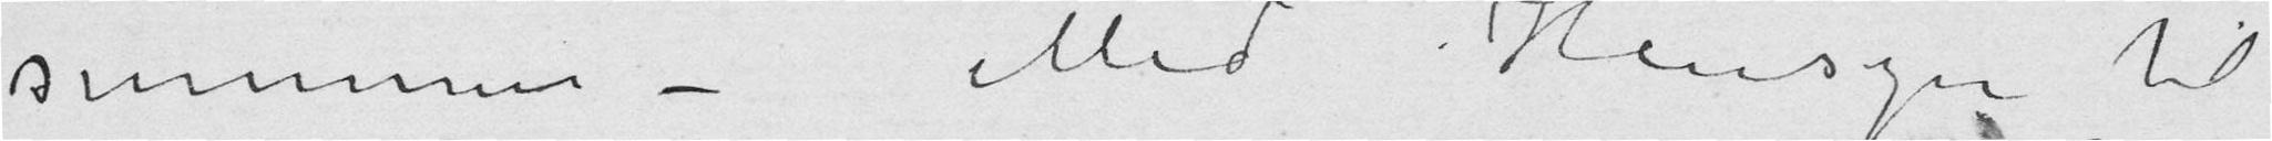summen – Med Hensyn til
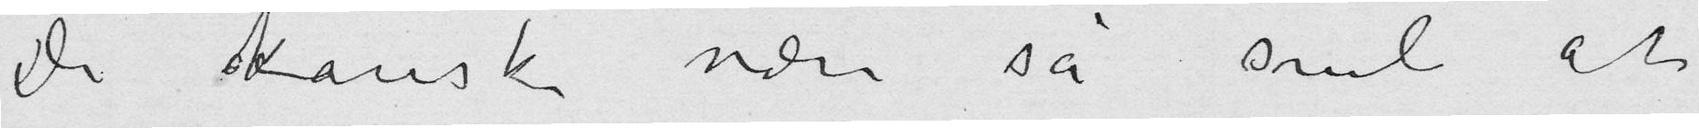De kanske være så snil at
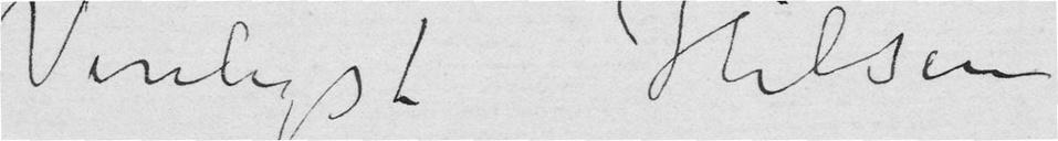Venligst Hilsen
